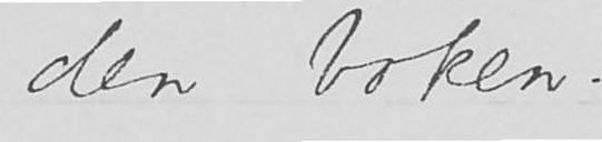den boken.
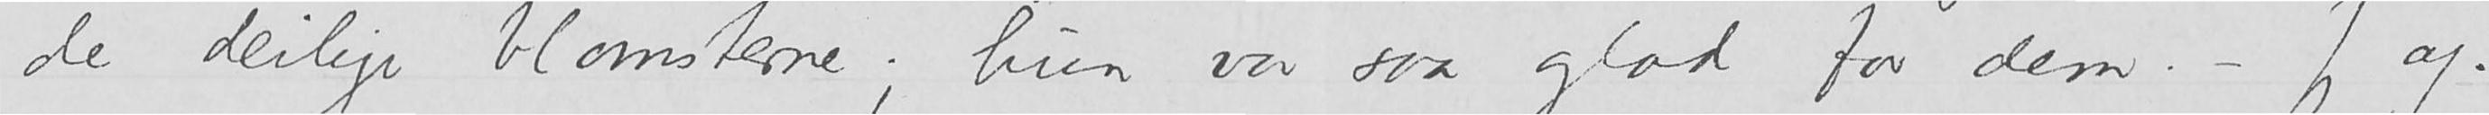de deilige blomsterne; hun var saa glad for dem. – Jeg og.
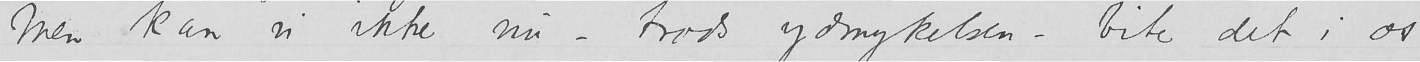Men kan vi ikke nu – trods ydmykelsen – bite det i os
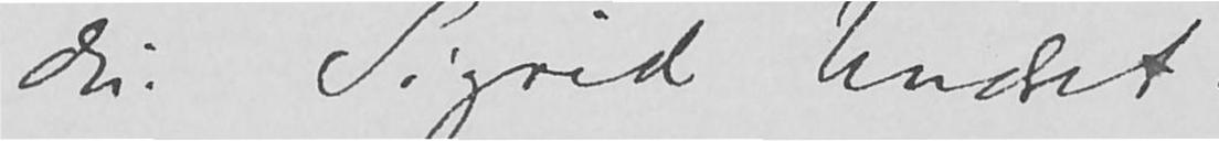Din Sigrid Undset.
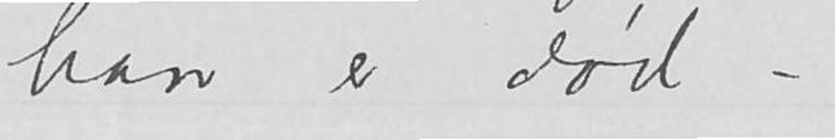han er død -.
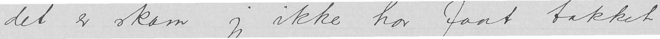det er skam jeg ikke har faat takket
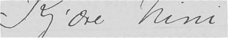Kjære Nini,
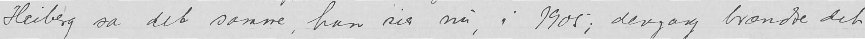Heiberg sa det samme, han sier nu, i 1905; dengang brændte
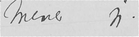Mener jeg.
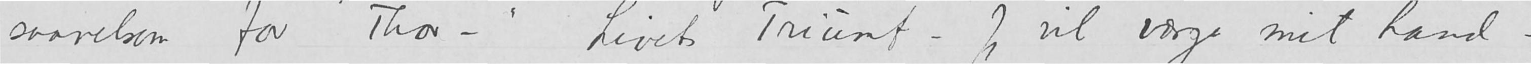Loke saavelsom for Thor –»  Livets Triumf – Jeg vil værge mit land –
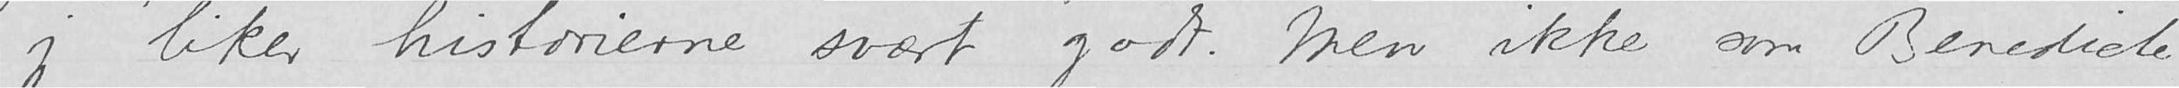– jeg liker historierne svært godt. Men ikke som Benedicte
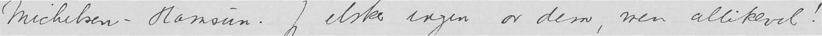Michelsen - Hamsun. Jeg elsker ingen av dem, men allikevel!
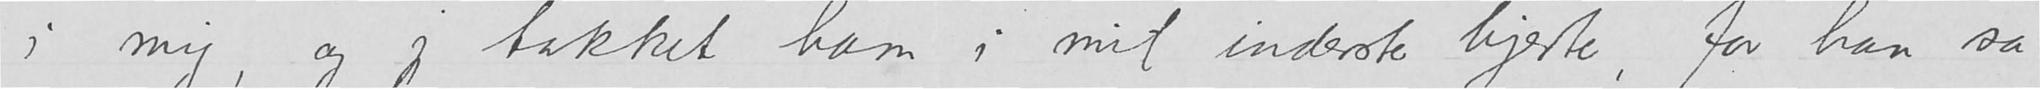det i mig, og jeg takket ham i mit inderste hjerte, for han sa
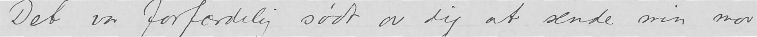Det var forfærdelig sødt av dig at sende min mor
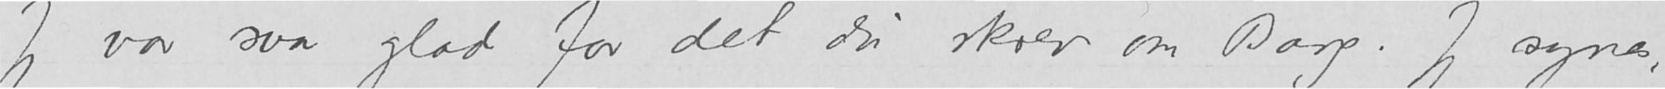Jeg var saa glad for det du skrev om Pago/Pogs. Jeg synes
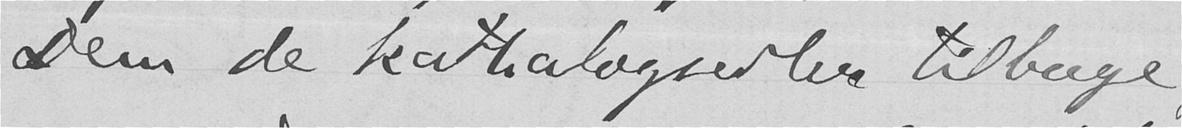Dem de kathalogsedler tilbage,
